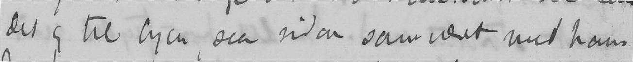det og til byen, saa siden samværet med ham.
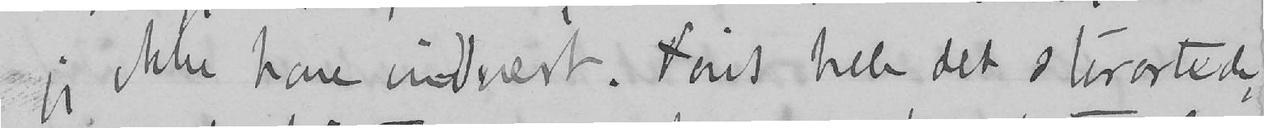jeg ikke have undvært. Først hele det storartede,
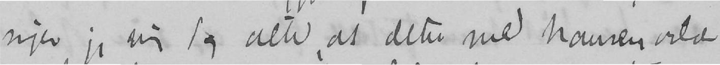siger jeg nu dog altid, at dette med Nansen vilde
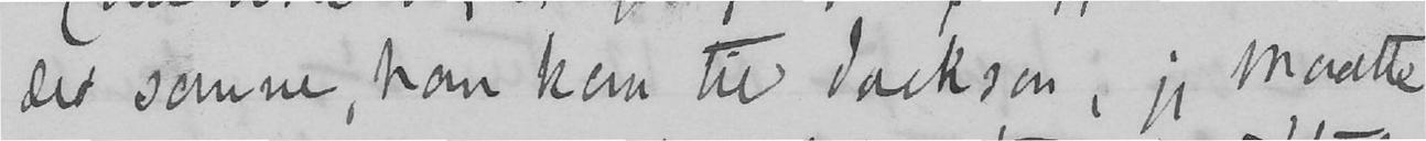det samme, han kom til Jackson, jeg maatte
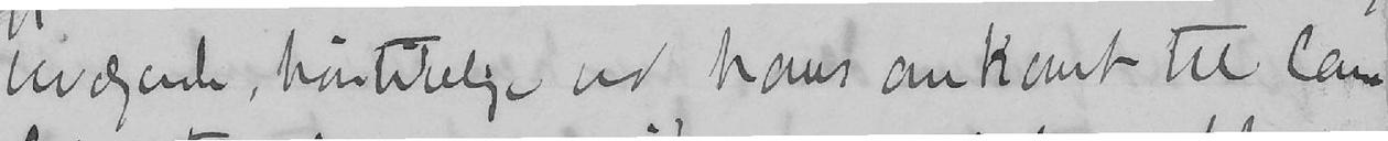bevægende, høitidelige ved hans ankomst til lan-
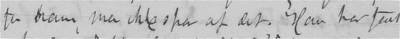for ham, men ikke spor af det. Han har faat
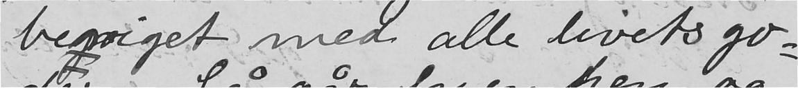beriget med alle livets go-
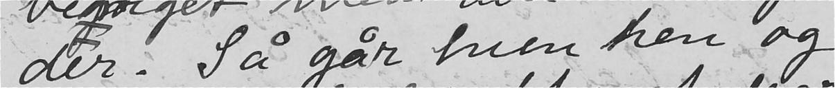der. Så går hun hen og
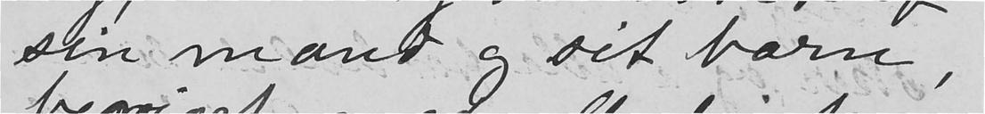sin mand og sit barn,
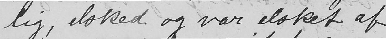lig, elsked og var elsket af
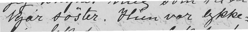kjær søster. Hun var lykke-
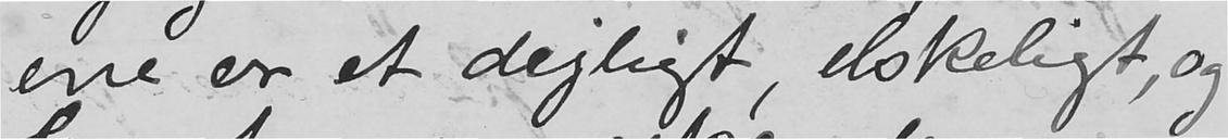ene er et dejligt elskeligt, og
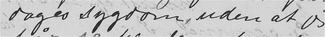dages sygdom, uden at jeg
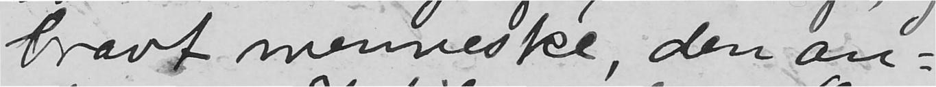bravt menneske, den an-
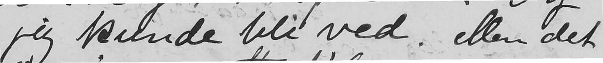jeg kunde bli ved. Men det
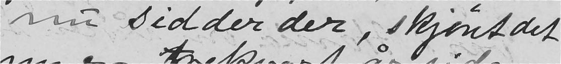nu sidder der, skjønt det
